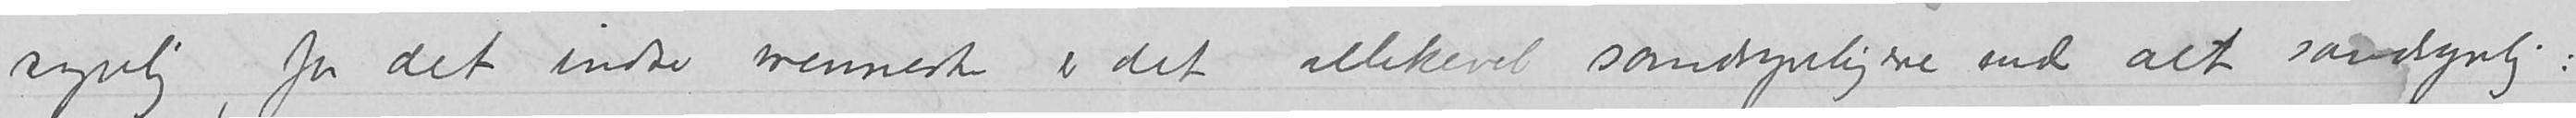synlig, for det indre menneske er det allikevel sandsynligere end alt sandsynlig:
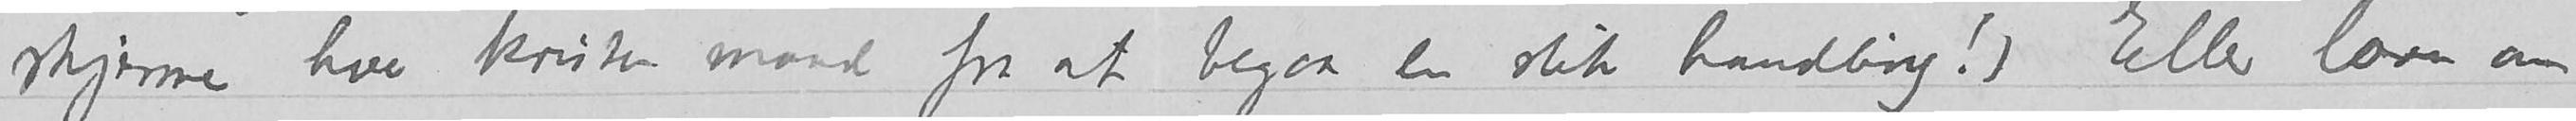skjermer hver kristen mand fra at begaa en slik handling!) Eller loven om
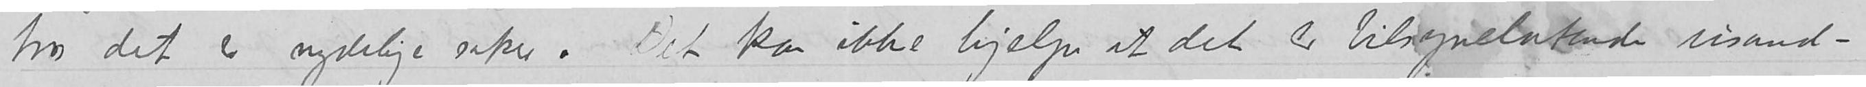tro det er nydelige saker. Det kan ikke hjelpe at det er tilsynelatende usand
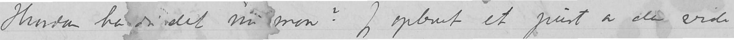Hvordan har du det nu mon? Jeg oplevet et pust av den vide
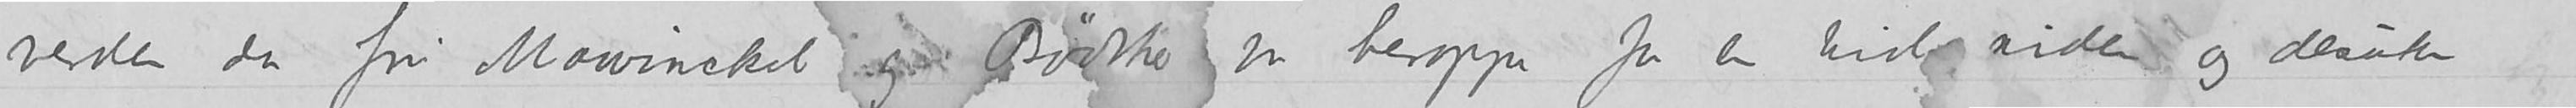verden da fru Mowinckel og Bødtker var heroppe for en tid siden og desuten
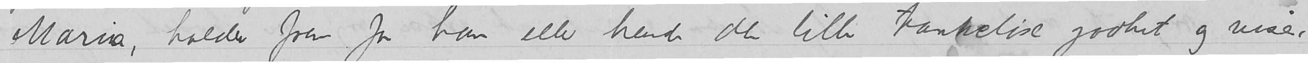Maria, holder frem for ham eller hende den lille tankeløse godhet og viser
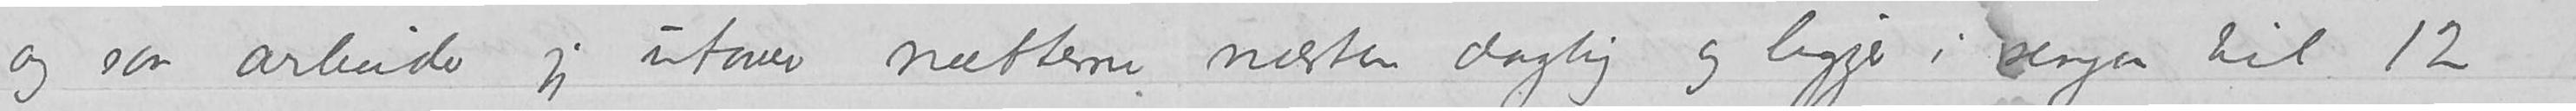og saa arbeider jeg utover nætterne nesten daglig og ligger i sengen til 12
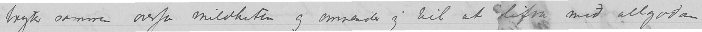bryter sammen overfor mildheten og omvender sig til at «lifva með allgoðan
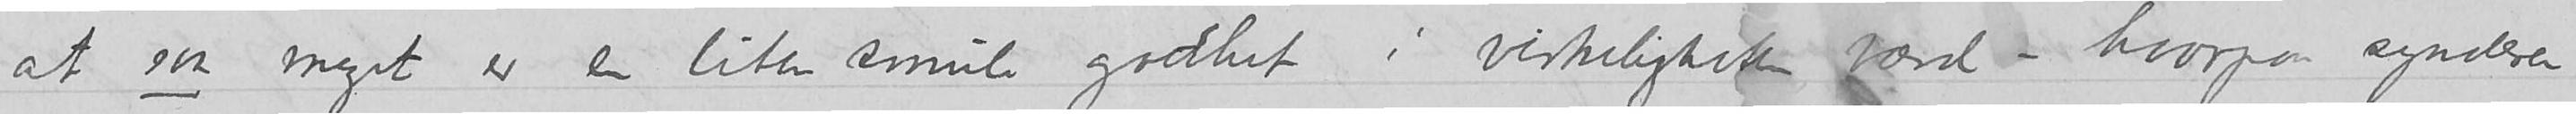at saa meget er en liten smule godhet i virkeligheten værd -hvorpaa synderen
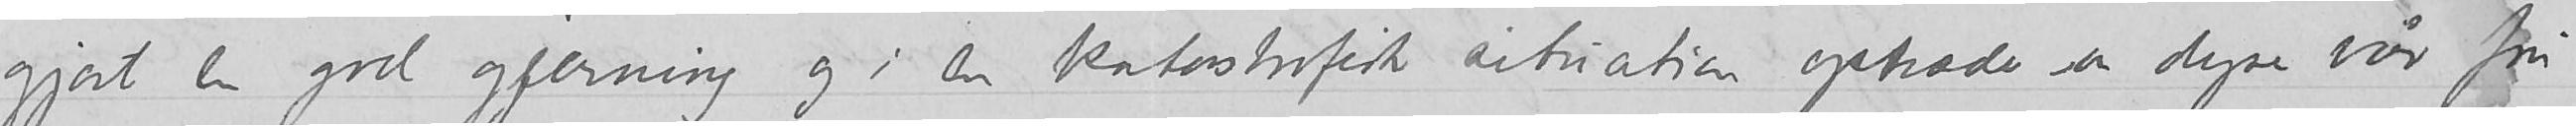gjort en god gjerning og i en katastrofisk situation optræder saa dyre vår fru
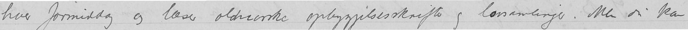hver formiddag og læser oldnorske opbyggelsesskrifter og lovsamlinger. Men du kan
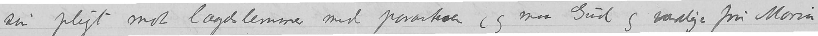sin pligt mot lægdslemmer med paranteser (og naar Gud og værdige fru Maria
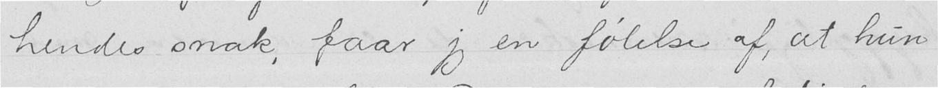hendes snak, faar jeg en følelse af, at hun
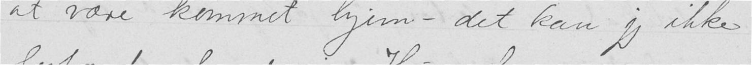at være kommet hjem — det kan jeg ikke
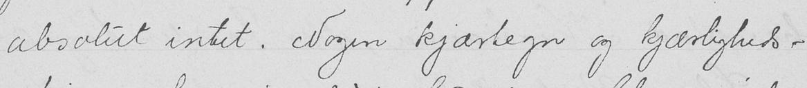absolut intet. Nogen kjærtegn og kjærligheds-
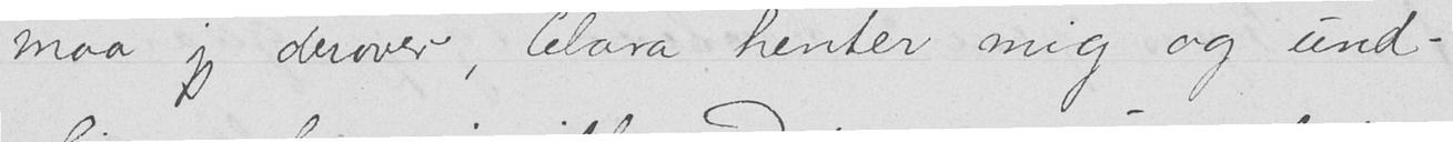maa jeg derover, Clara henter mig og und-
